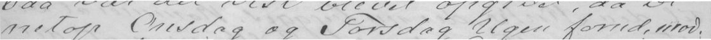netop Onsdag og Torsdag Ugen forud, mod-
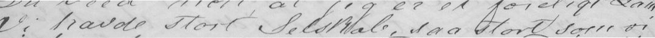Vi havde stort Selskab, saa stort som vi
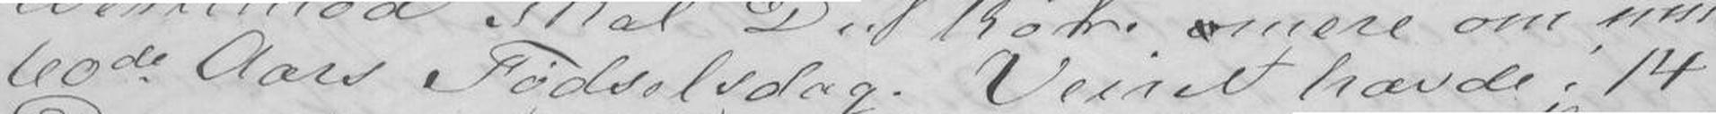60de Aars Fødselsdag. Veiret havde i 14
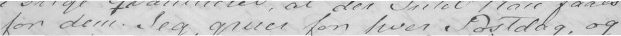for dem. Jeg gruer for hver Postdag, og
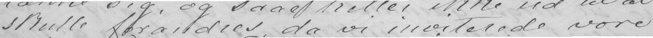skulle forandres, da vi inviterede vore
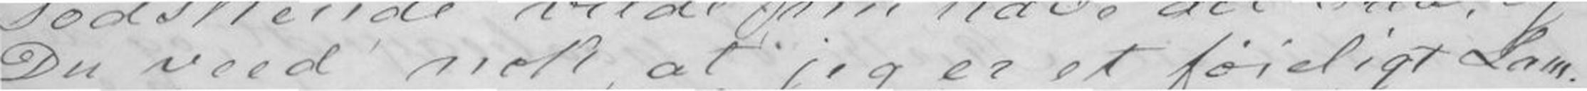Du veed nok at jeg er et føieligt Lam.
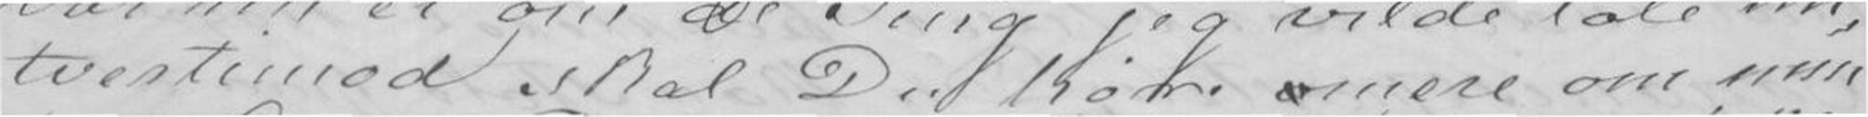tvertimod skal Du høre mere om min
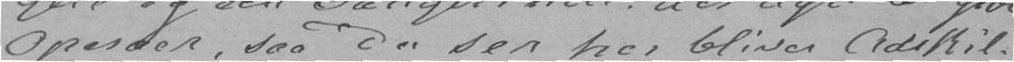Operaer, saa Du ser her bliver Adskil-
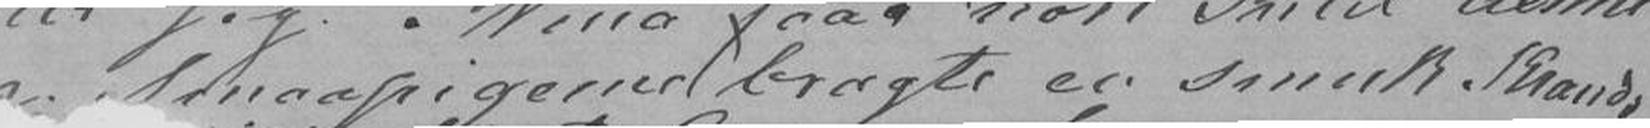Smaapigerne bragte en smuk Krands
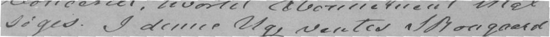siges I denne Uge ventes Skougaard
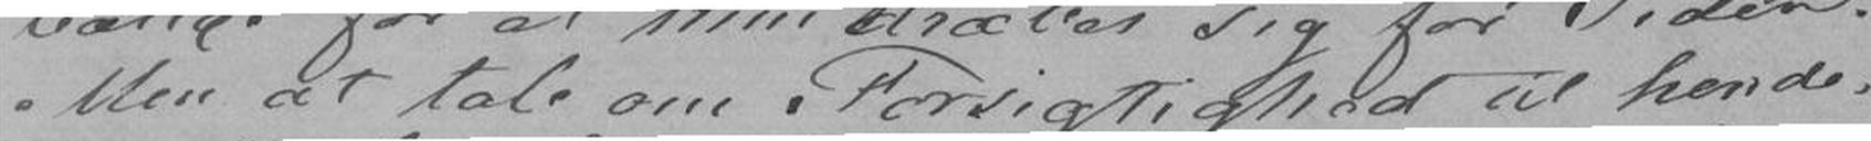Men at tale om Forsigtighed til hende
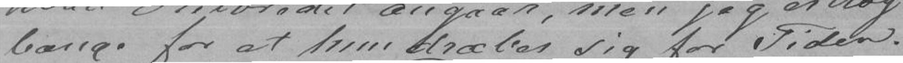bange for at hun dræber sig for Tiden.
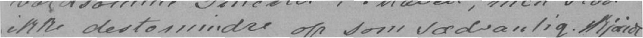ikke destomindre op som sædvanlig skjøndt
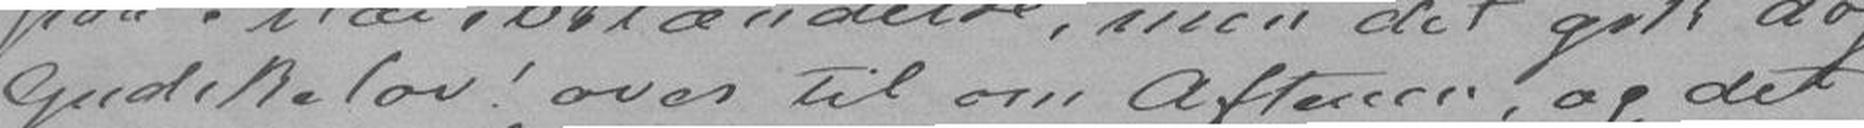Gudskelov! over til om Aftenen, og det
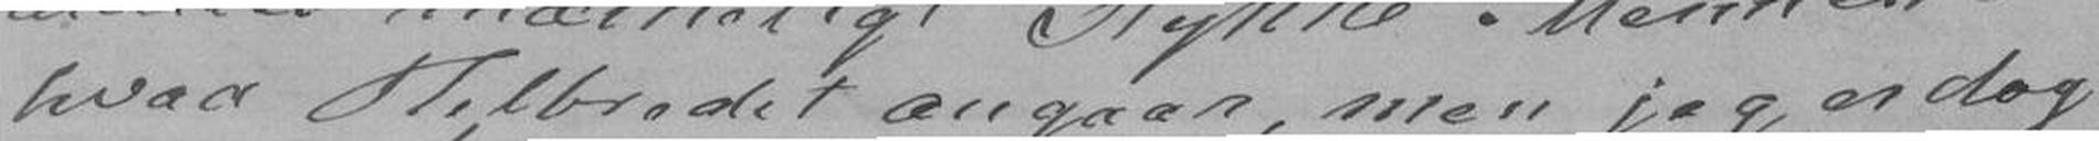hvad Helbredet Angaar, men jeg er dog
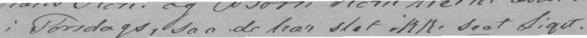i Torsdag, saa de har slet ikke seet Liget.
